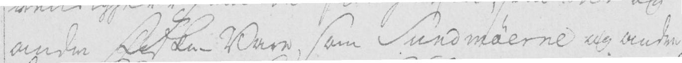andre Fiske-Vare, som Sundmøerne og andre
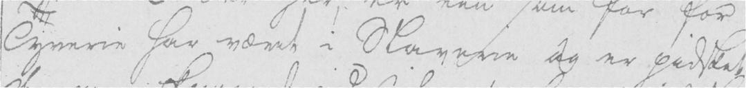Tyverie har været i Slaverie og er pidsket
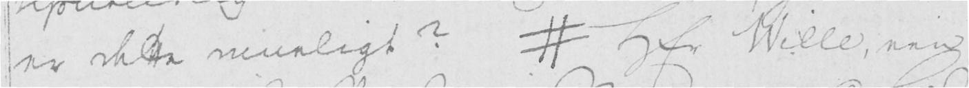er dette mueligt? # Hr Wille, een
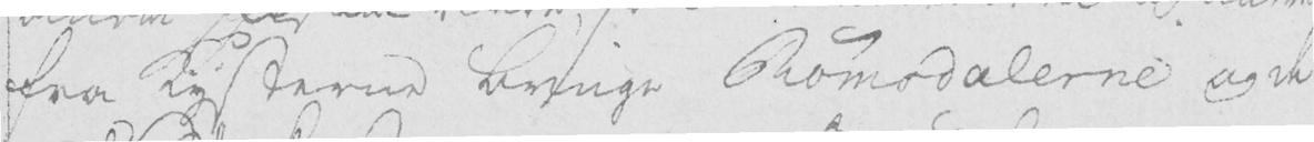fra Kysterne bringe Romsdalerne og de
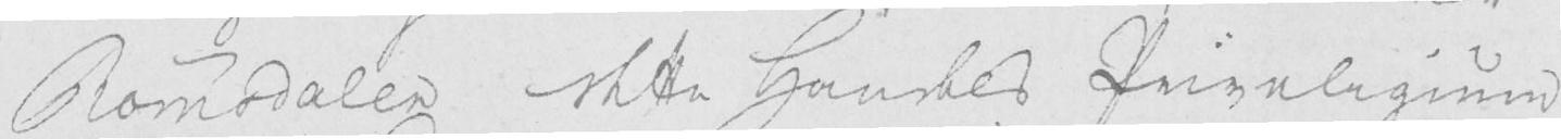Romsdalen dette Handels Privelegium
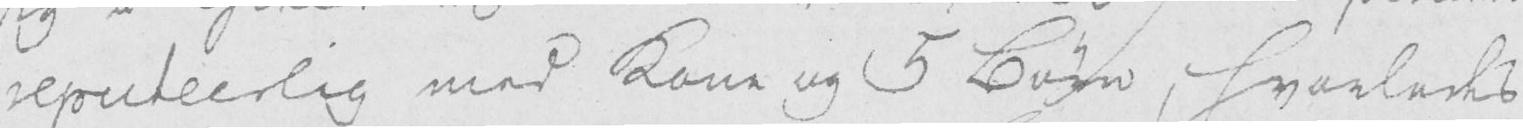reputerlig med Kone og 5 Børn, hvorledes
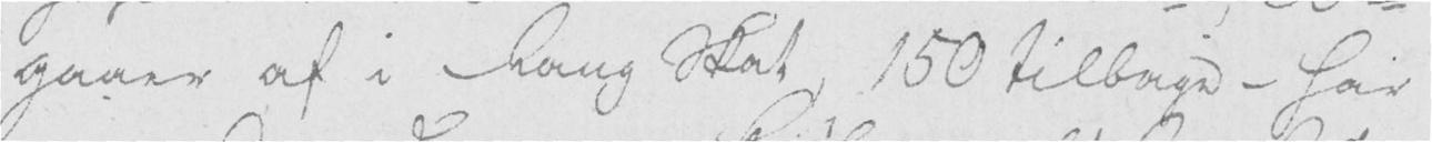gaaer af i kong Skat, 150 tilbage - har
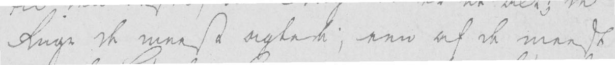Rige de meest agtede; een af de meest
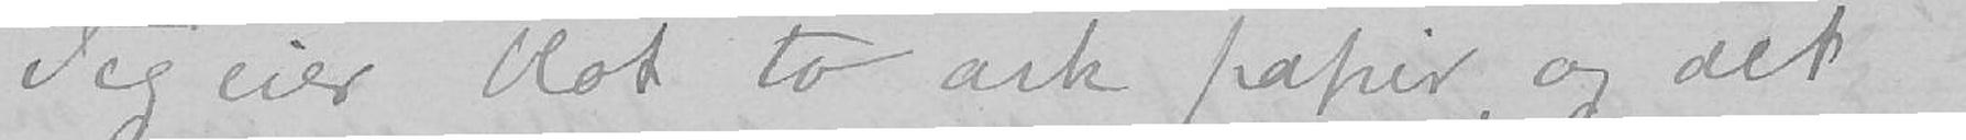Jeg eier blot to ark papir, og det
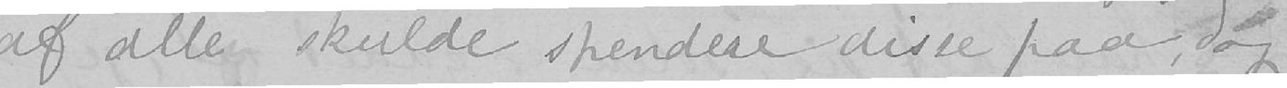af alle skulde spendere disse paa, dog
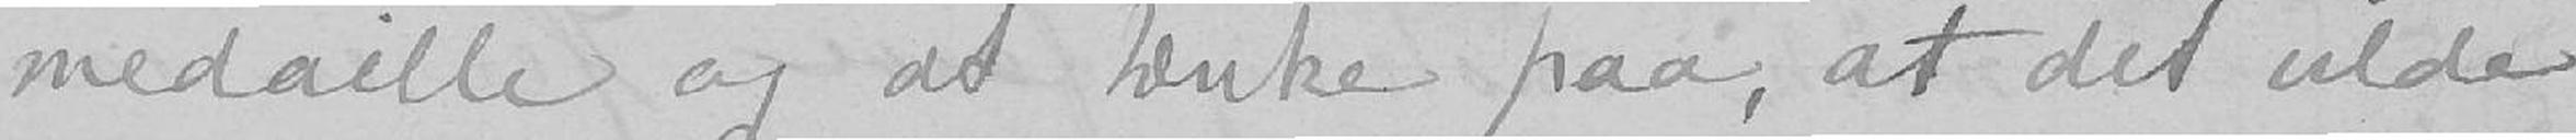medaille og at tænke paa, at det
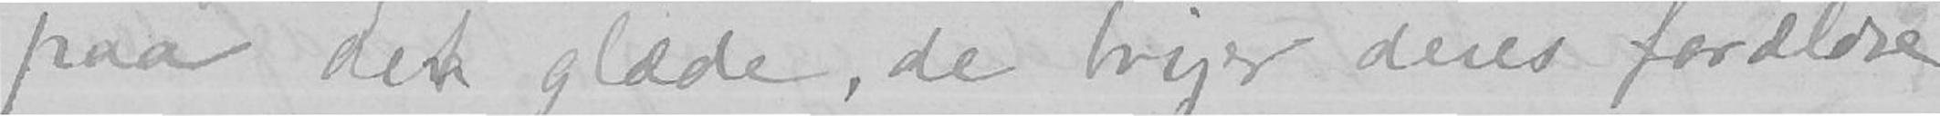paa den glæde, de bringer deres forældre
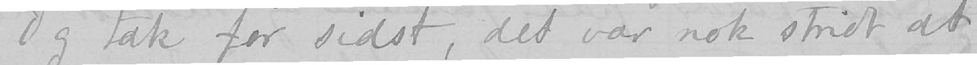Og tak for sidst, det var nok stridt at
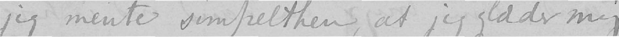jeg mente simpelthen, at jeg glæder mig
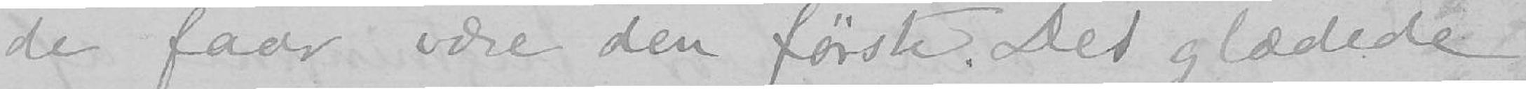de faar være den første. Det glædede
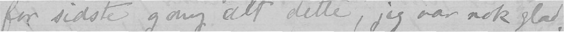for sidste gang alt dette, jeg var nok glad,
